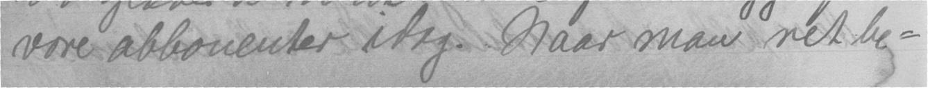vore abbonenter idag. Naar man ret be-
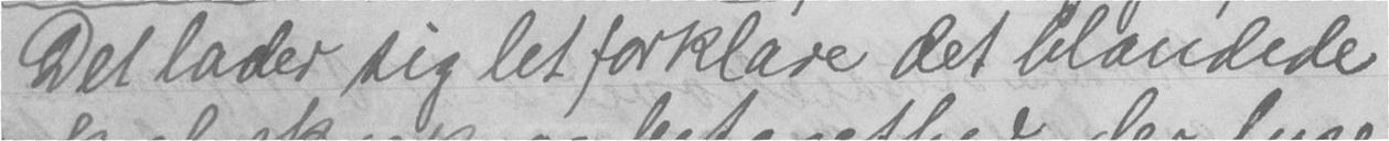Det lader sig let forklare det blandede
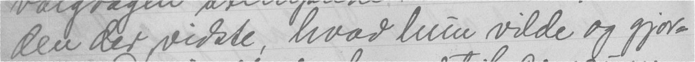den der vidste, hvad hun vilde og gjor-
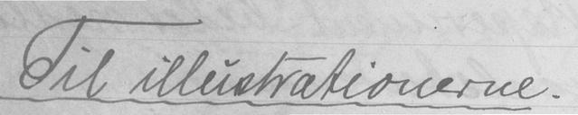Til illustrationerne.
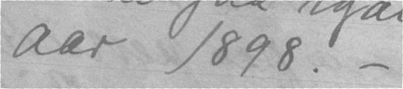aar 1898. -
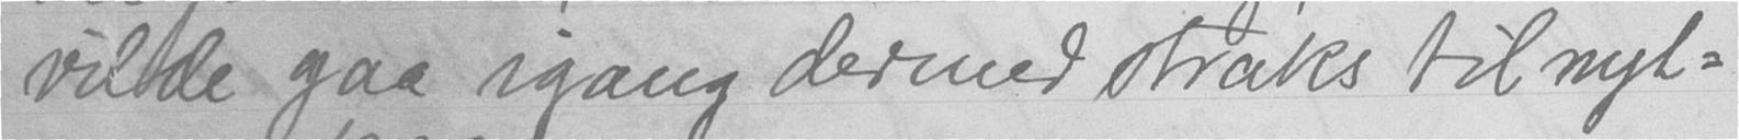villde gaa igang dermed straks til nyt-
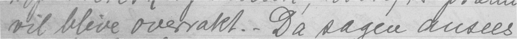vil blive overrrakt. - Da sagen ansees
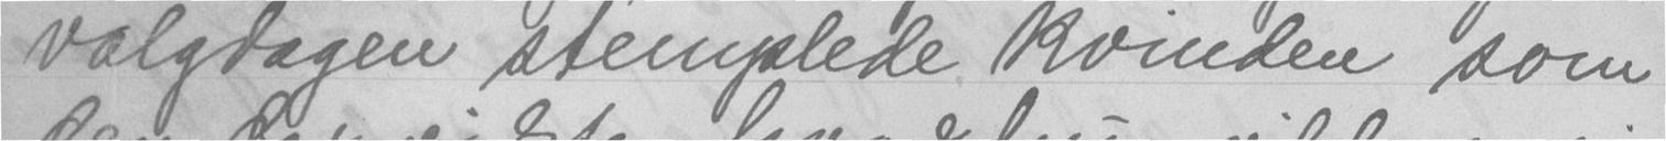valgdagen stemplede kvieden som
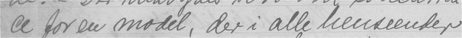ce for en model, der i alle henseender
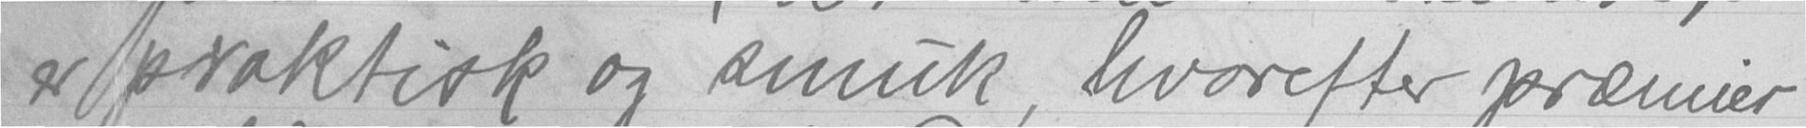er praktisk og smuk, hvorefter præmier
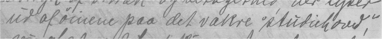ud af øinene paa det vakre "studiehoved,"
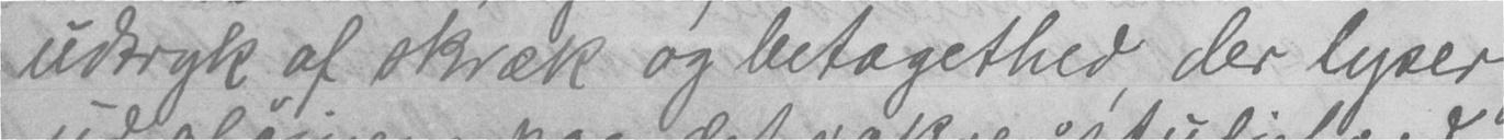udtryk af skræk og betagethed, der lyser
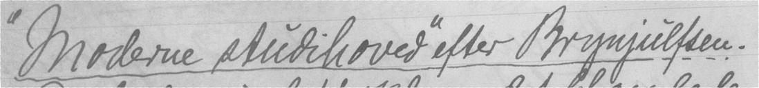"Moderne studihoved" efter Brynjulfsen.
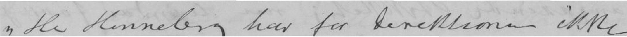"Hr Henneberg har for Direktionen ikke
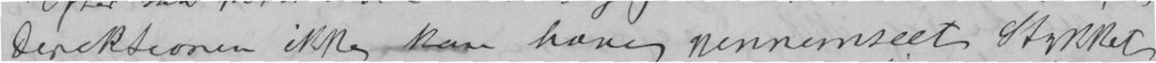Direktionen ikke, kan have gjennemseet Stykket,
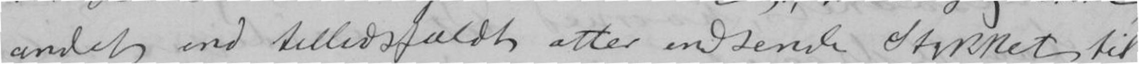andet end tillidsfuldt atter indsende Stykket til
